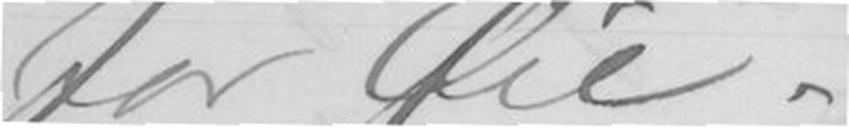for Øie.
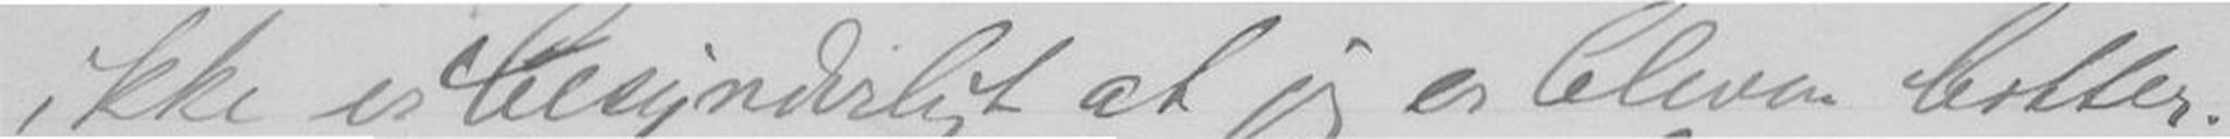ikke er besynderligt at jeg er bleven bitter.
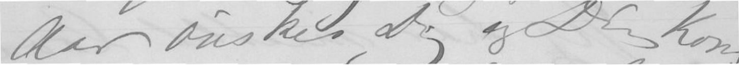Aar ønskes Dig og Din Kone
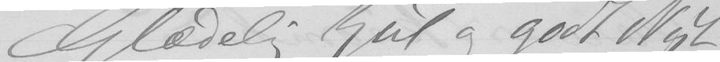Glædelig Jul og godt Nyt
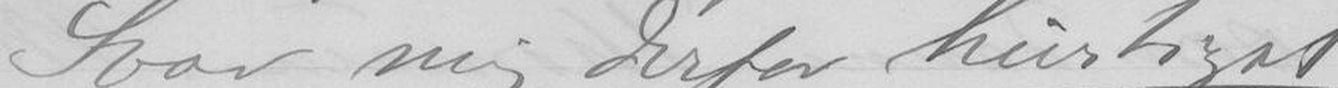Svar mig derfor hurtigst
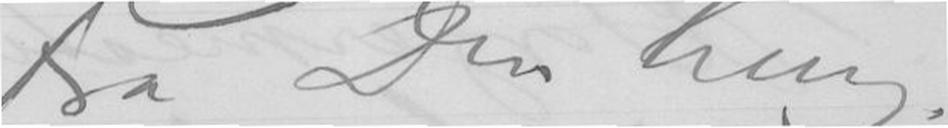fra Din heng.
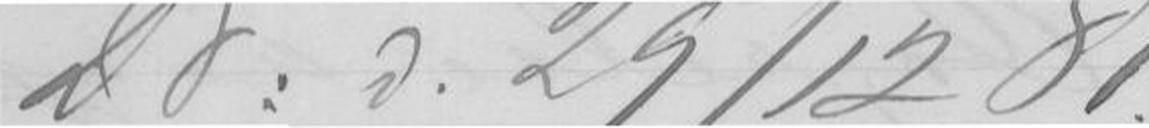Dr. d. 29/12 81
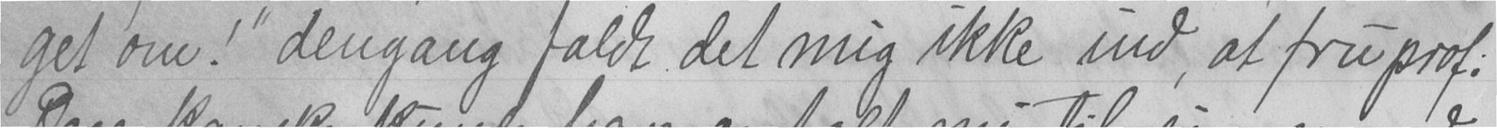get om!" dengang faldt det mig ikke ind, at fru prof.
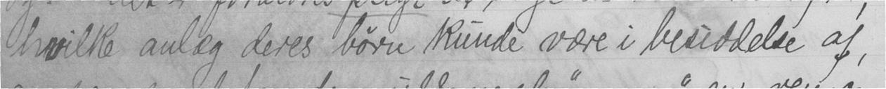hvilke anlæg deres børn kunde være i besiddelse af,
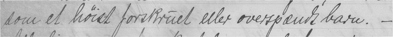som et høist forskruet eller overspændt barn. -
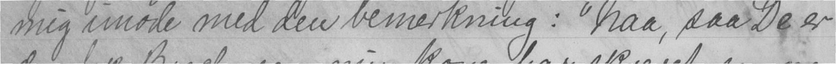mig imøde med den bemerkning: "Naa, saa De er
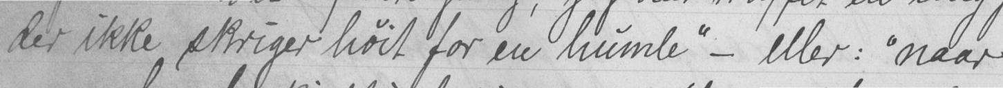der ikke skriger høit for en humle" - eller: "naar
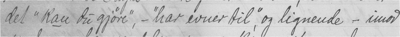det "kan du gjøre", -" har evner til", og lignende - imod
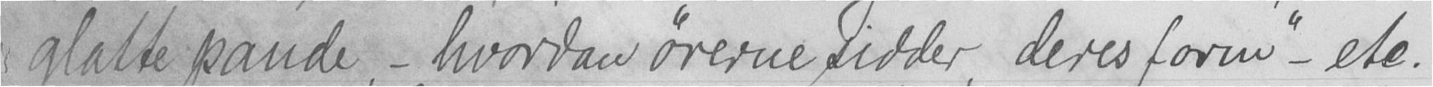glatte pande, - hvordan ørerne sidder, deres form" - etc.
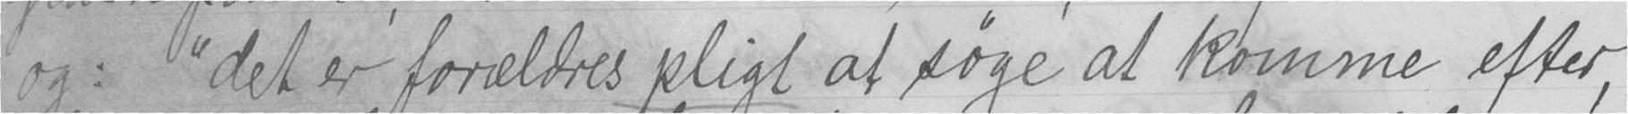og: "det er forældres pligt af søge at komme efter
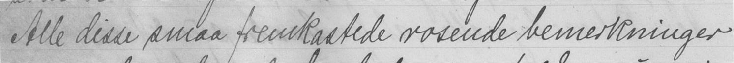Alle disse smaa fremkastede rosende bemerkninger
